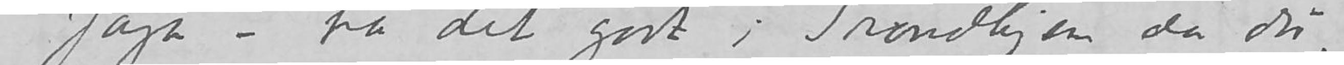Jaja – ha det godt i Trondhjem da du.
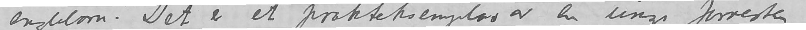englebarn.  Det er et prakteksemplar av en unge forresten –
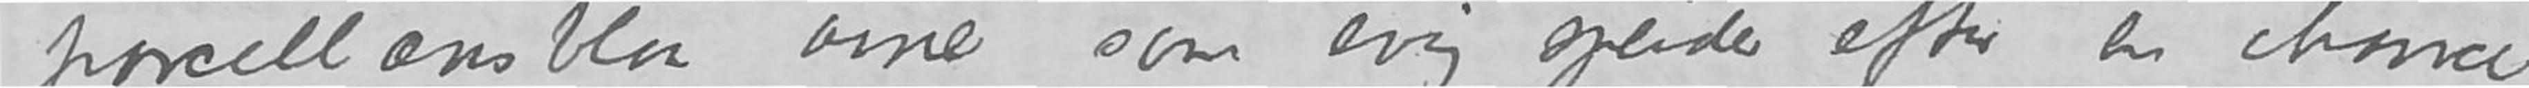porcellænsblaa øine som evig speider efter en chance –
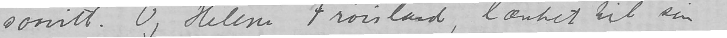saavidt. Og Helene Frøisland, lænket til sin
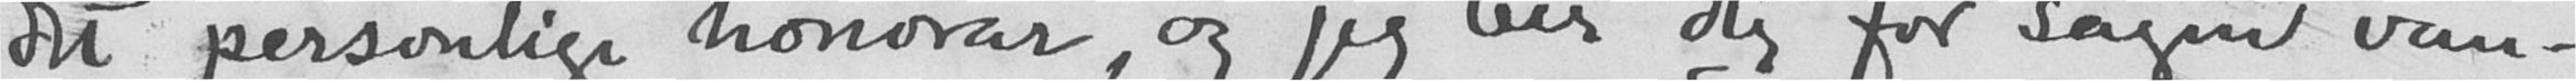det personlige honorar, og jeg ber dig for sagen van-
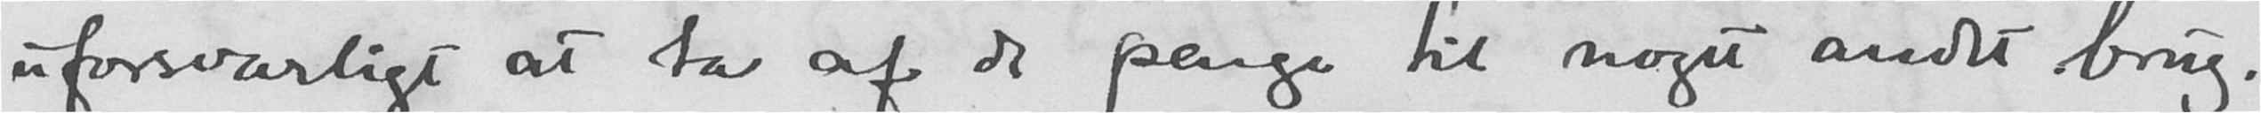uforsvarligt at ta af de penge til noget andet brug..
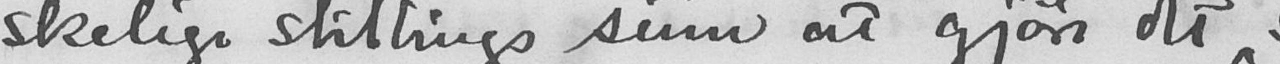skelige stillings sum at gjøre det
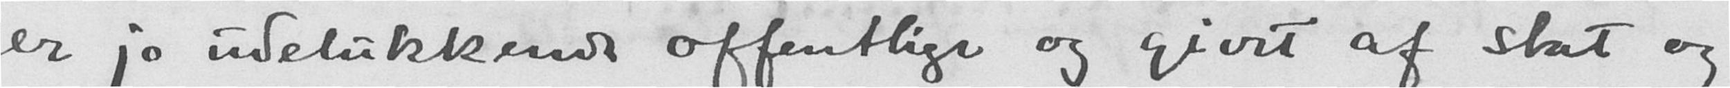er jo udelukkende offentlige og givet af stat og
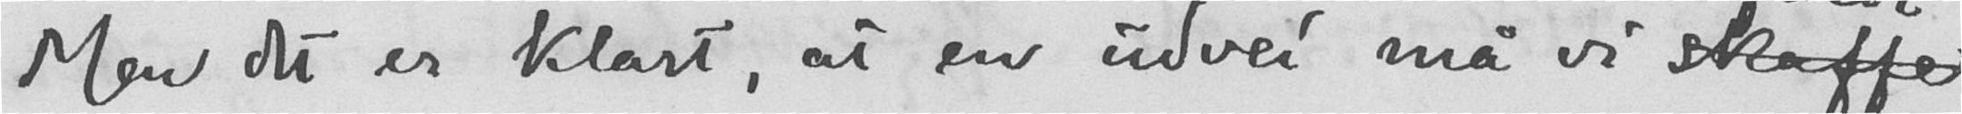Men det er klart, at en udvei må vi skaffe
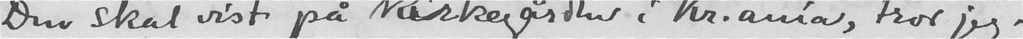Den skal vist på kirkegården  i Kr. ania, tror jeg.
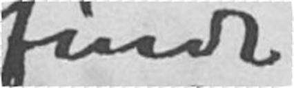finde
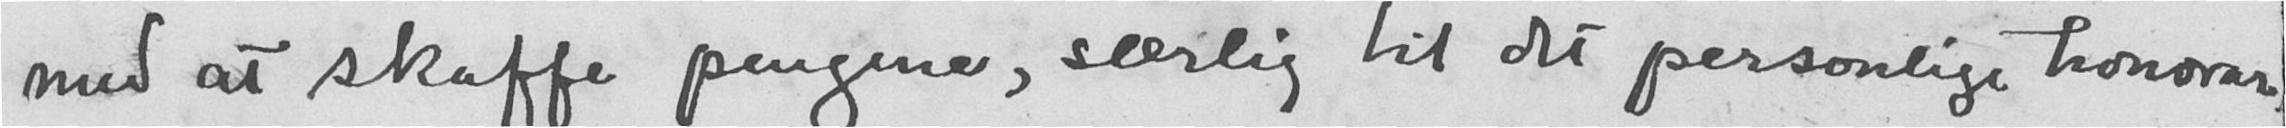med at skaffe pengene, særlig til det personlige honorar.
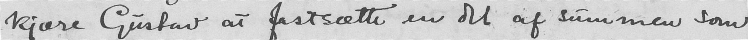kjære Gustav at fastsætte en del af summen som
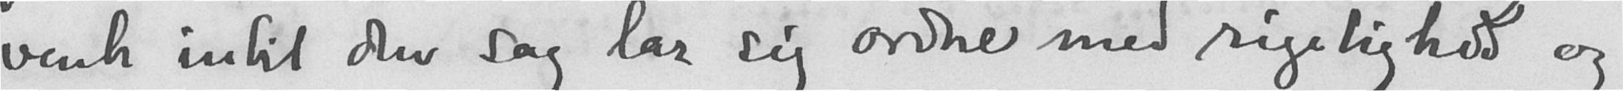vente intil den sag lar sig ordne med rigelighed og
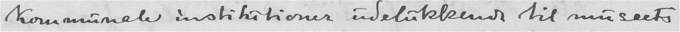kommunale institutioner udelukkende til mussets
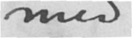med
